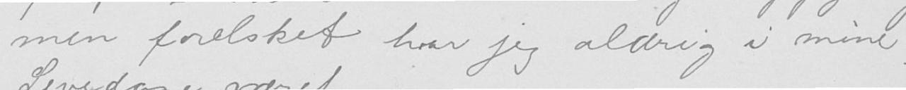men forelsket har jeg aldrig i mine
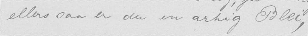ellers saa er du en artig Blei,
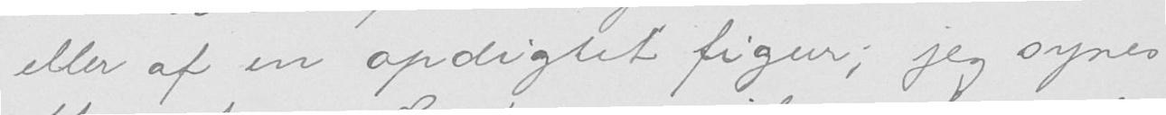eller af en opdigtet figur; jeg synes
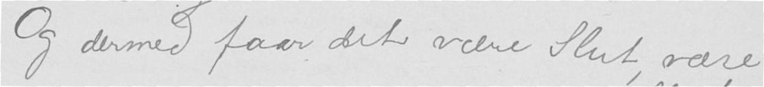Og dermed faar det være Slut, være
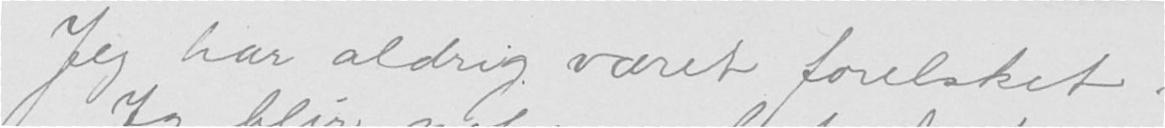Jeg har aldrig været forelsket.
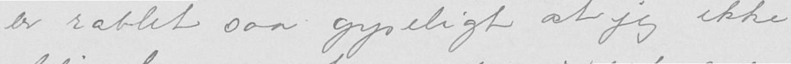er rablet saa gyseligt at jeg ikke
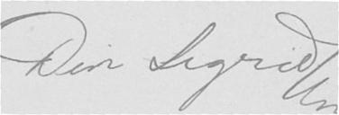Din Sigrid
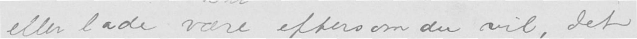eller lade være eftersom du vil, det
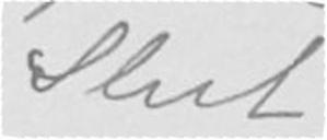Slut,
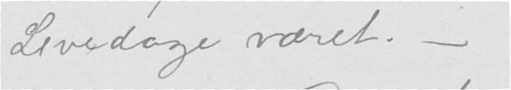Levedage været. -
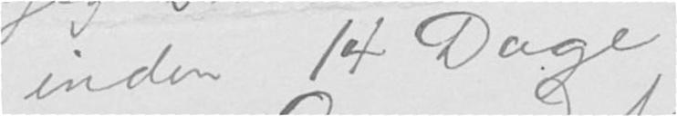inden 14 Dage
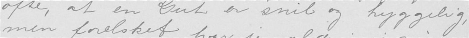ofte, at en Gut er snil og hyggelig,
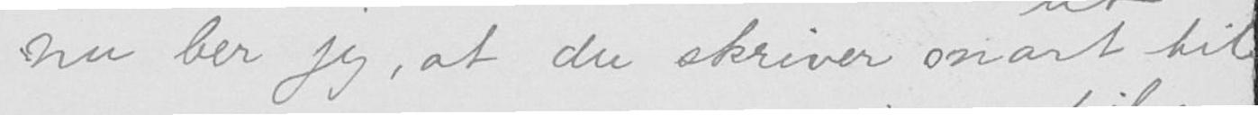nu ber jeg, at du skriver snart til
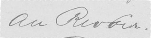Au Revoir.
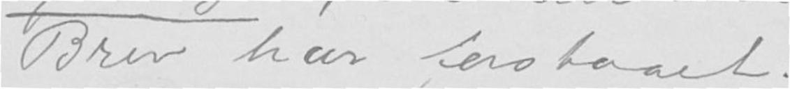Brev har forstaaet.
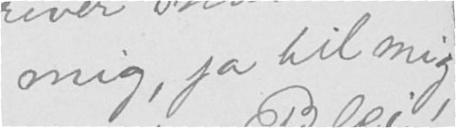mig, ja til mig,
